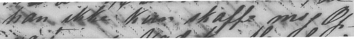han ikke kan skaffe mig Op-
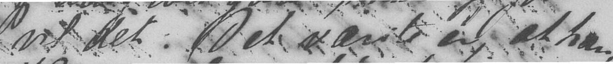vil det. Det værste er, at han
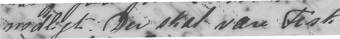nedligt. Der skal være Fisk
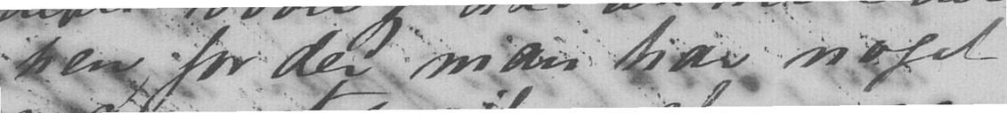hen, for der man har noget
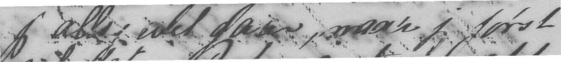jeg alligevel faar, naar jeg først
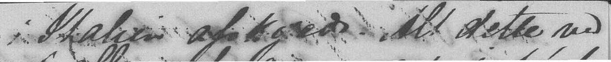i Italien afskyede. Alt dette ved
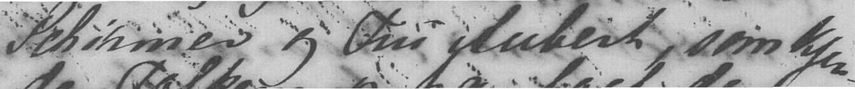Schirmer og Fru Aubert, som kjen-
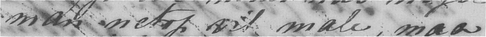man netop vil male, maa
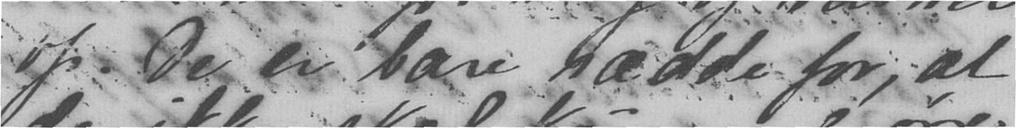op. De er bare rædde for, at
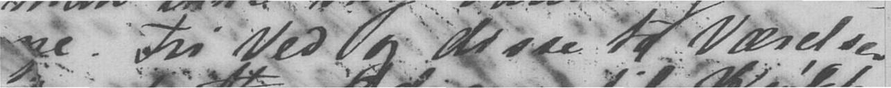ne. Fri Ved og disse to Værelser
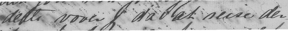dette vover jeg da at reise der-
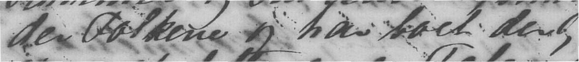der Folkene og har boet der,
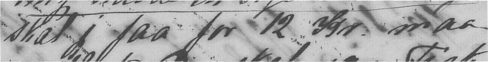skal jeg faa for 12 Kr. maa-
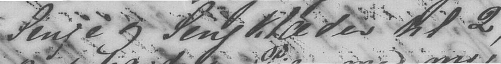Senge og Sengklæder til 2,
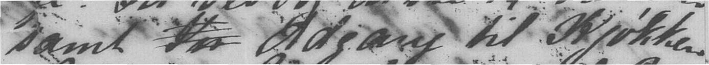samt Jir Adgang til Kjøkken
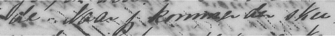de. Naar jeg kommer der sku-
